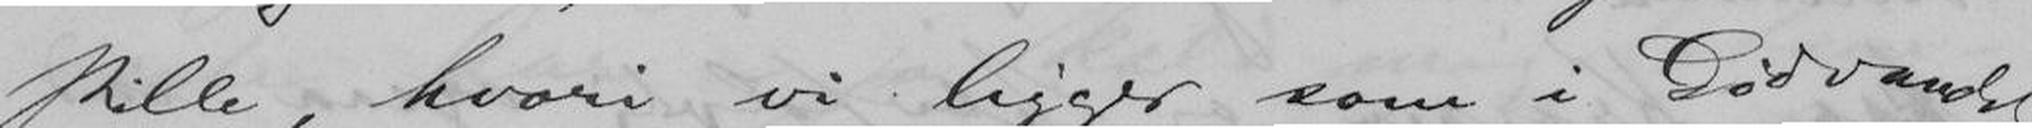stille, hvori vi ligger som Dødvandet.
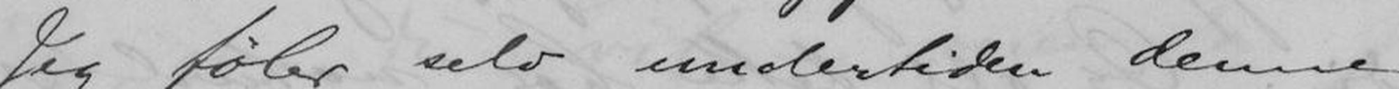Jeg føler selv undertiden denne
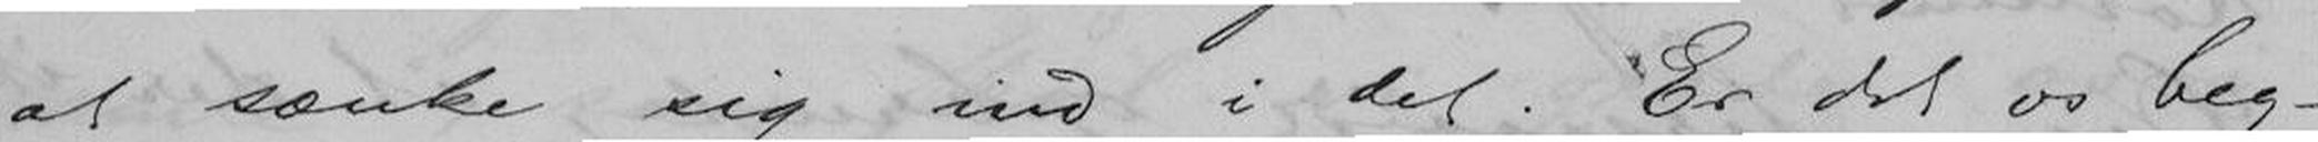at sænke sig ind i det. Er det os beg-
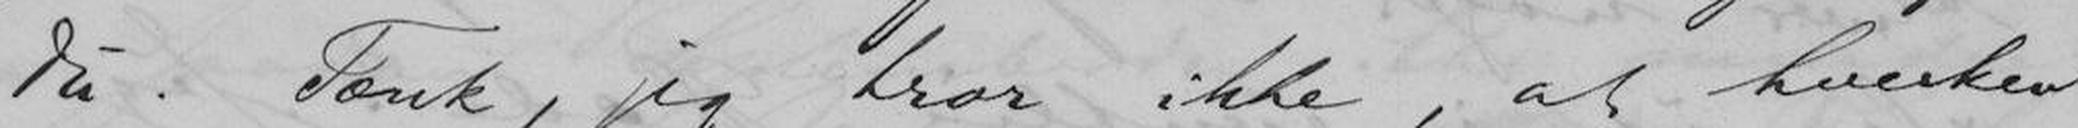Du. Tænk, jeg tror ikke, at hverken
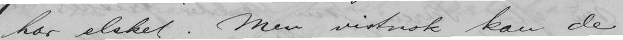har elsket. Men vistnok kan de
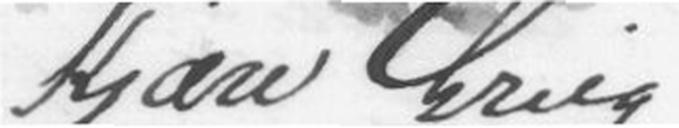Kjære Grieg
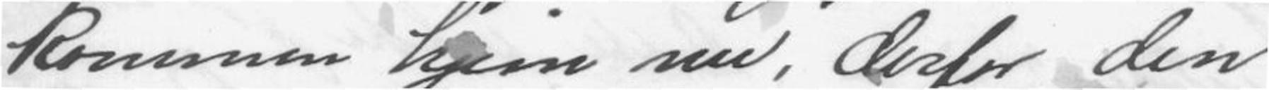kommen hjem nu, derfor den
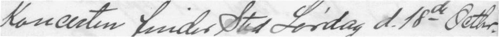Koncerten finder Sted Lørdag d. 18de Octbr.
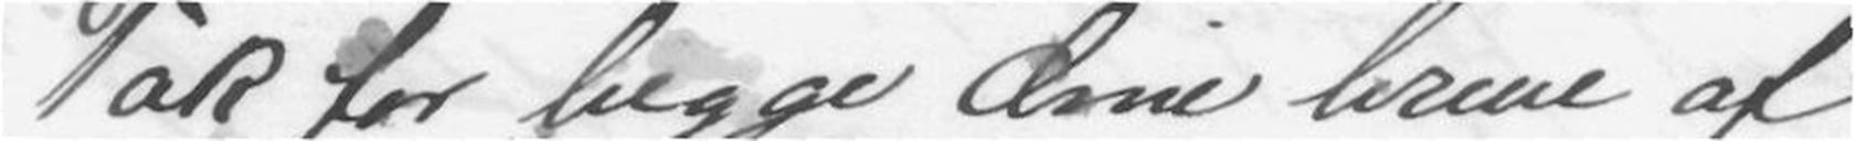Tak for begge dine breve af
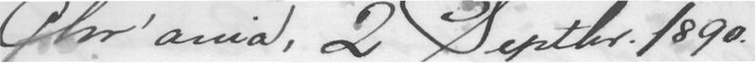Chr'ania, 2 Septbr. 1890.
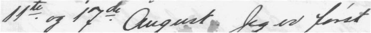11teog 17de August. Jeg er først
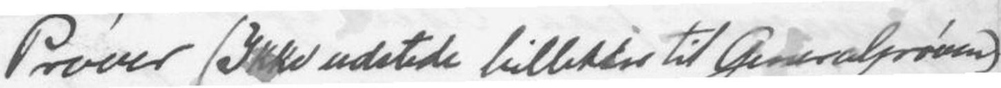Prøver (Ikke ustede billetter til Generalprøven)
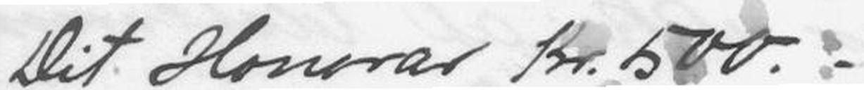Dit Honorar Kr. 500.-
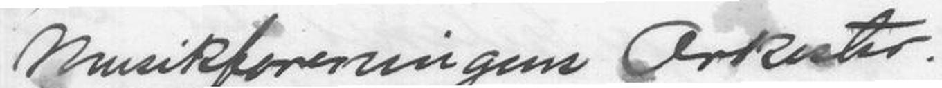Musikforeningens Orkester.
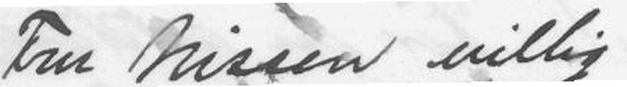Fru Nissen villig
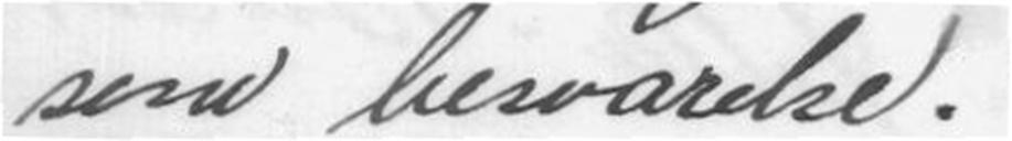sene besvarelse.
